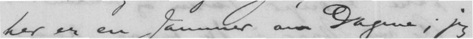her er en Jammer om Dagene; jeg
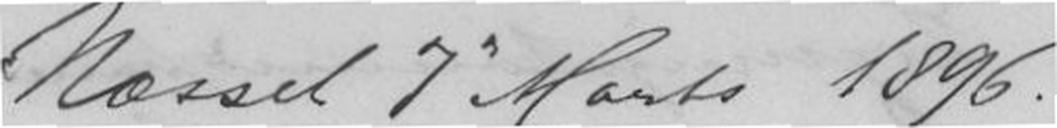Næsset 7de Marts 1896.
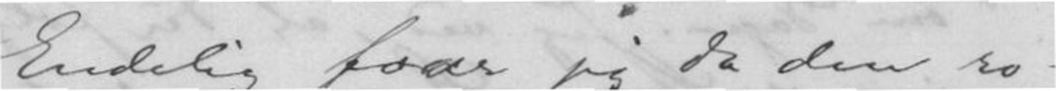Endelig faar jeg da den ro-
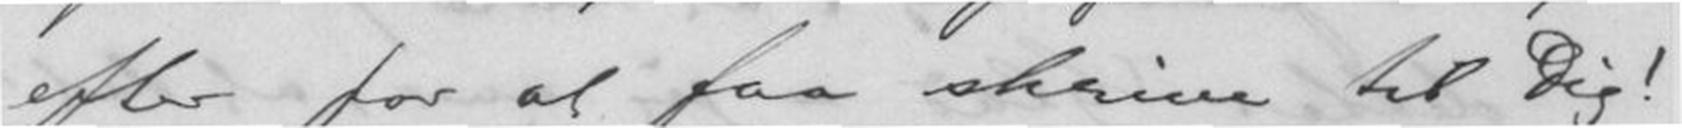efter for at faa skrive til Dig!
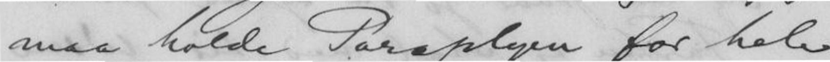maa holde Paraplyen for hele
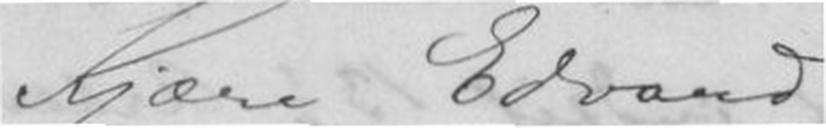Kjære Edvard!
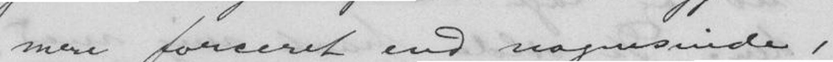mere forseret end nogensinde,
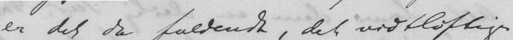er det da fuldendt, det vidtløftige
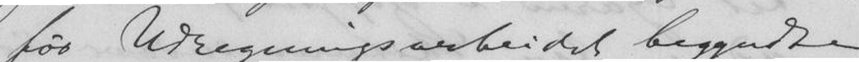før Udregningsarbeidet begyndte,
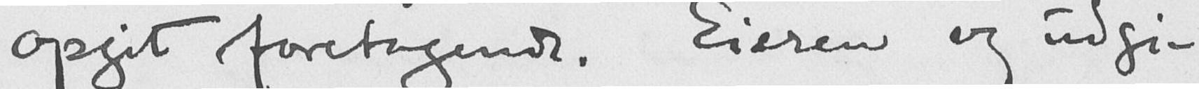opgit foretagende. Eieren og udgi-
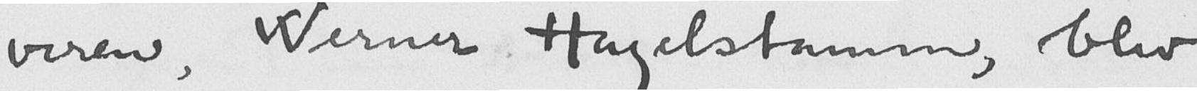veren, Werner Hagelstamm, blev
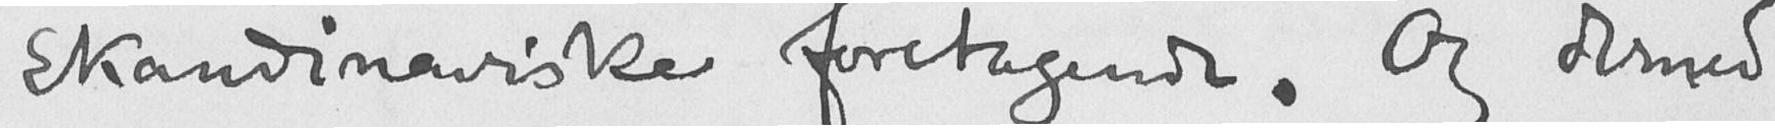skandinaviske foretagende. Og dermed
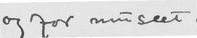og for museet.
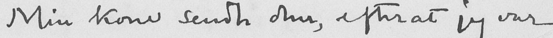Min kone sendte dem, efterat jeg var
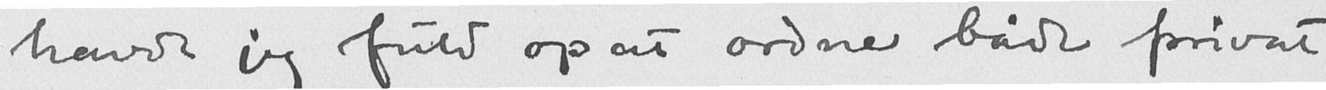havde jeg fuldt op at ordne både privat
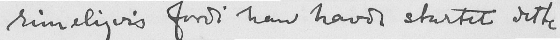rimeligvis fordi han havde startet dette
